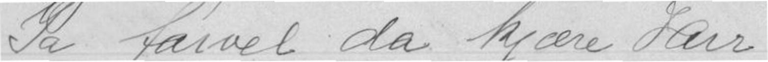Ja farvel da kjære Herr
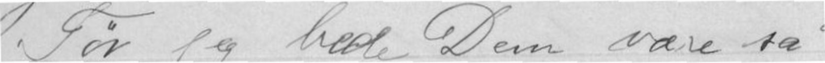Tør jeg bede Dem være så
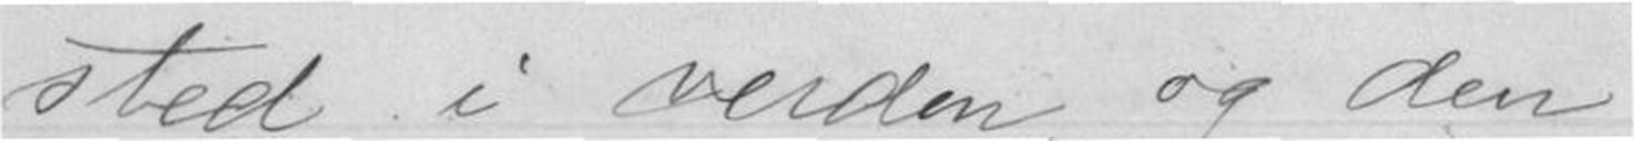sted i verden, og den
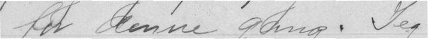for denne gang. Jeg
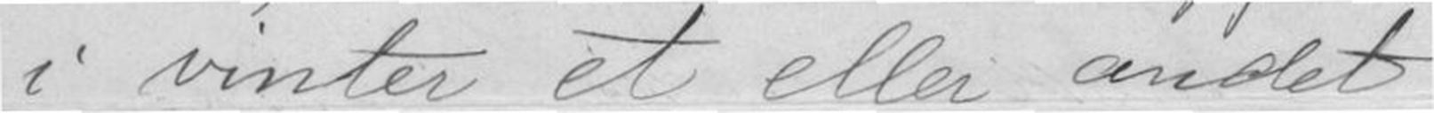i vinter et eller andet
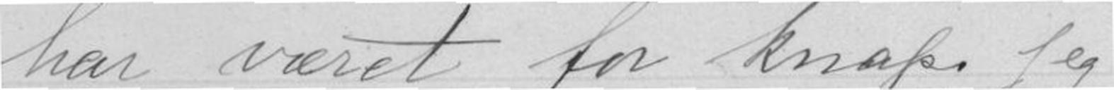har været for knap; jeg
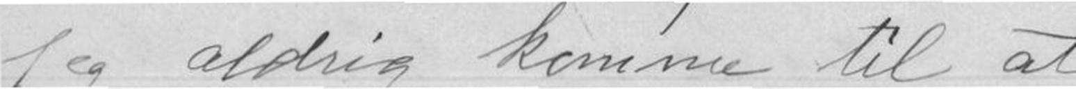jeg aldrig komme til at
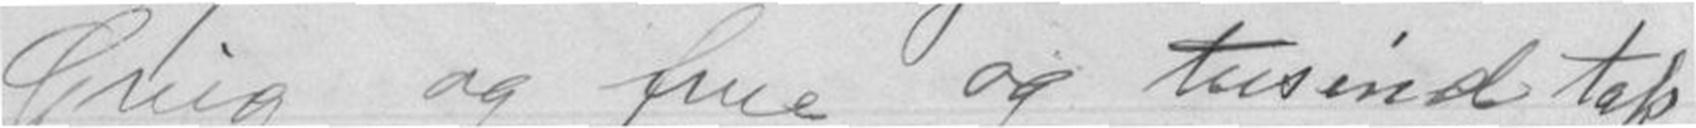Grieg og frue, og tusinde tak
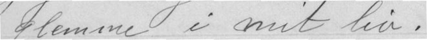glemme i mit liv.
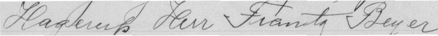Hagerup, Herr Frantz Beyer
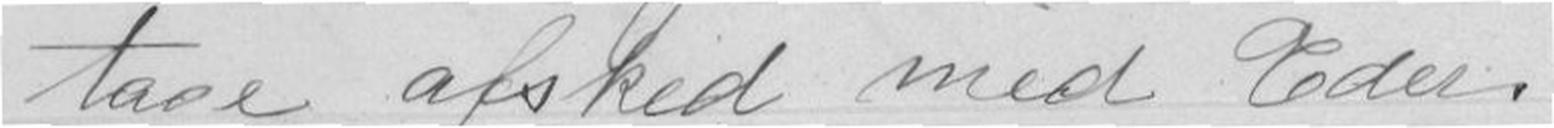tage afsked med Eder.
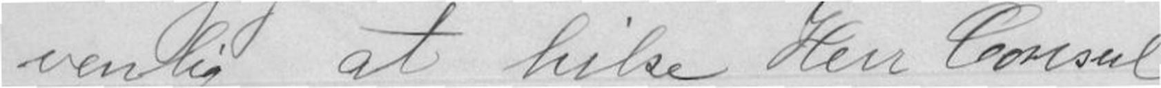venlig at hilse Herr Consul
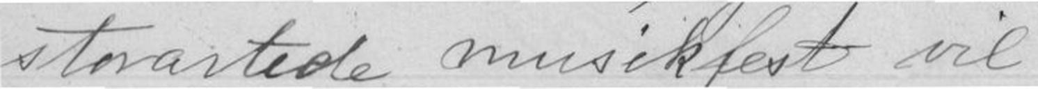storartede musikfest vil
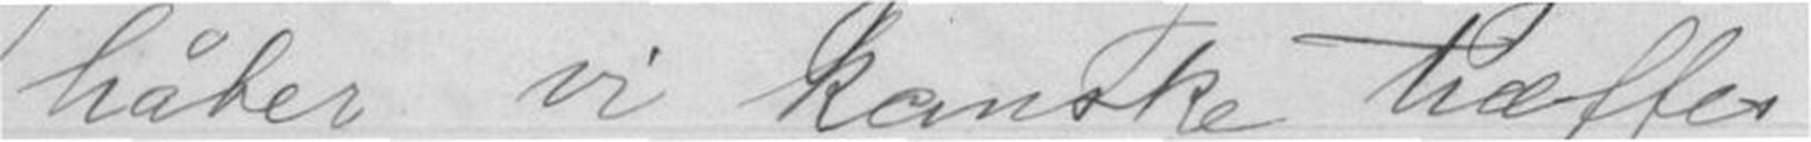håber, vi kanske træffes
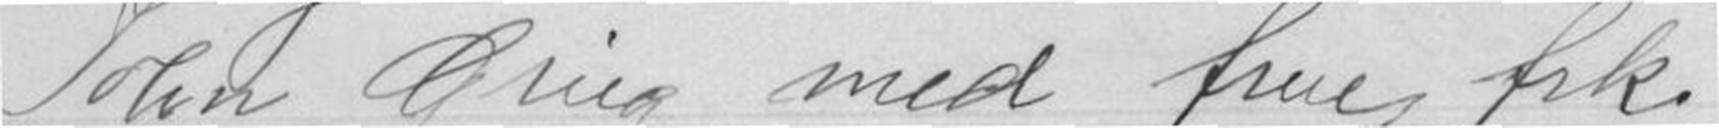John Grieg med frue, frk
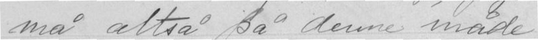må altså på denne måde
